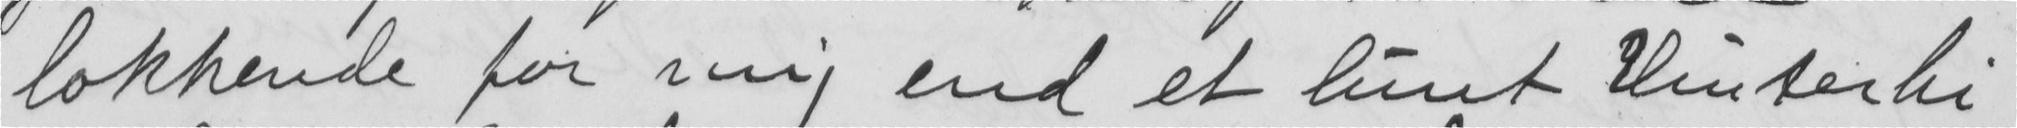lokkende for mig end et lunt Vinterhi
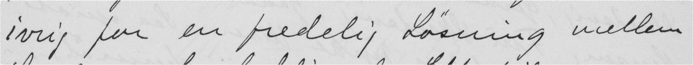ivrig for en fredelig Løsning mellem
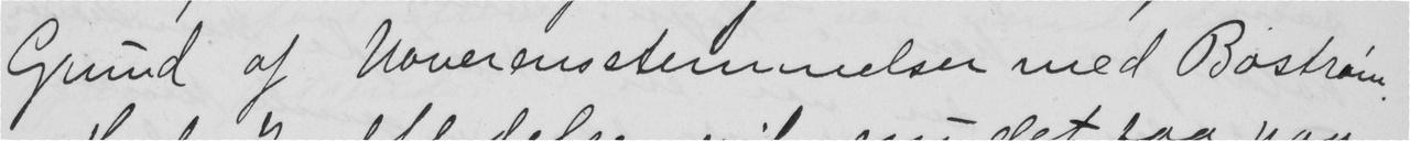Grund af Uoverensstemmelser med Bostrøm.
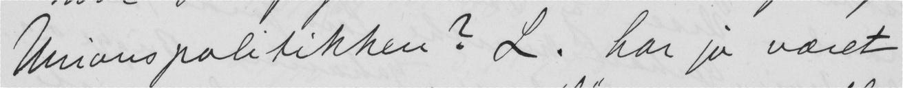Unionspolitikken? L. har jo været
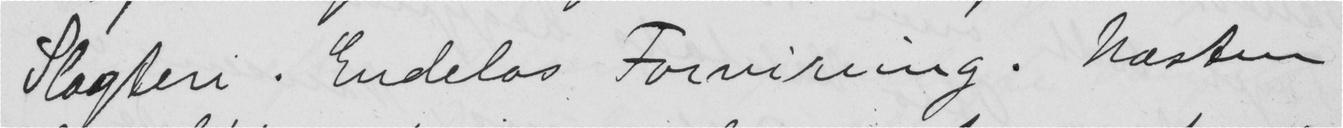Slagteri. Endeløs Forvirring. Næsten
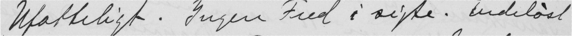Ufatteligt. Ingen Fred i sigte. Endeløst
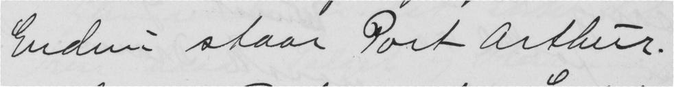Endnu staar Port Arthur.
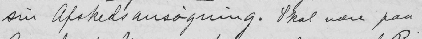sin Afskedsansøgning! Skal være paa
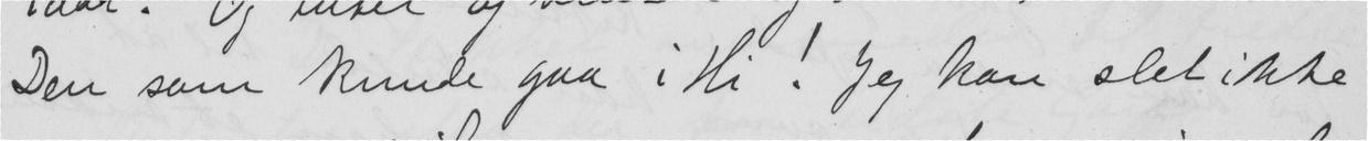Den som kunde gaa i Hi! Jeg kan slet ikke
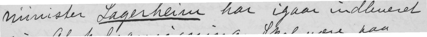minister Lagerheim har igaar indleveret
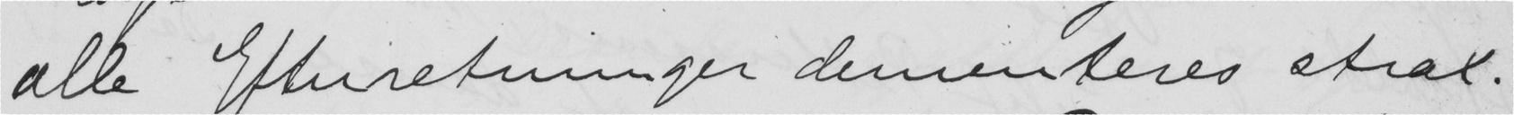alle Efterretninger dementeres strax.
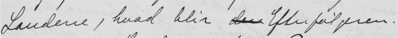Landene, hvad blir  Efterfølgeren.
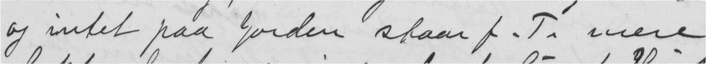og intet paa Jorden staar f.T. mere
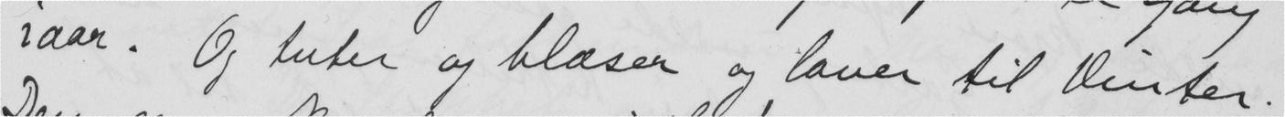iaar. Og tuter og blæser og laver til Vinter.
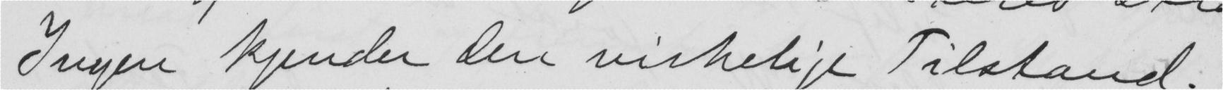Ingen kjender den virkelige Tilstand.
